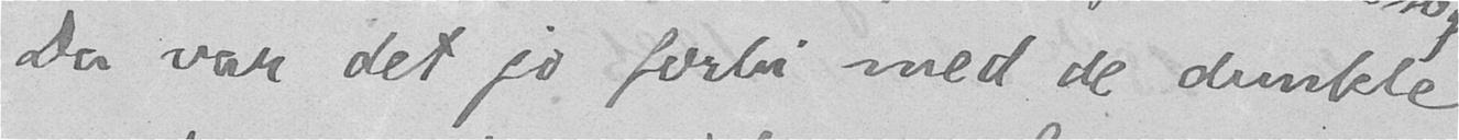Da var det jo forbi med de dunkle
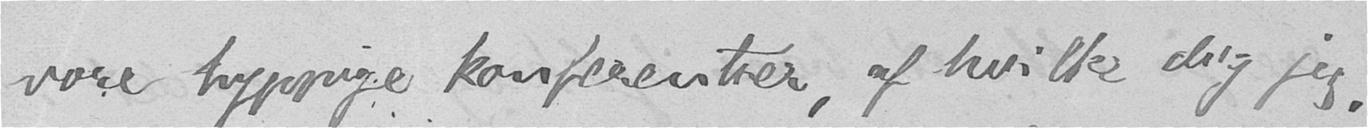vore hyppige konferentser, af hvilke dig jeg,
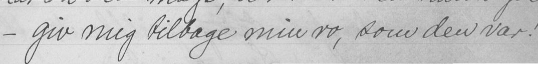- giv mig tilbage min ro, som den var!
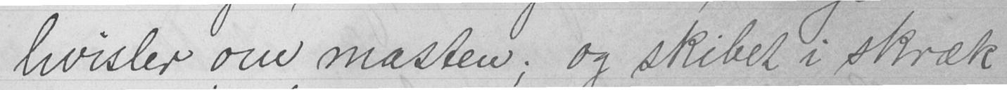hvisler om masten; og skibet i skræk
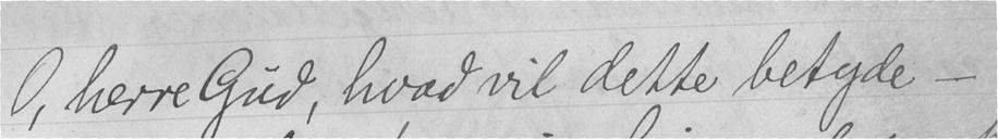O, herre Gud, hvad vil dette betyde -
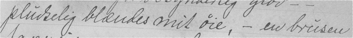pludselig blændes mit øie, - en brusen
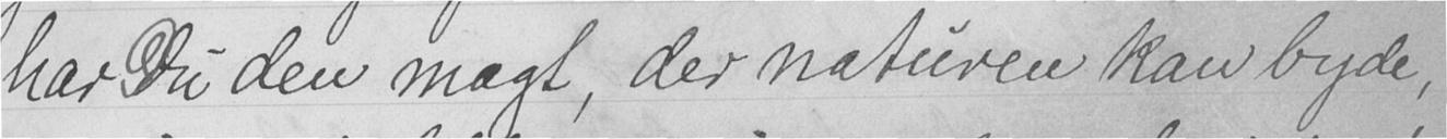har Ddu den magt, der natuven kan byde,
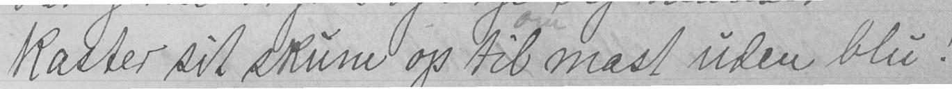kaster sit skum op til mast uden blu!
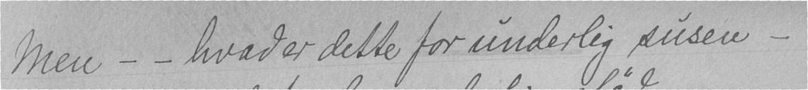Men - - hvad er dette for underlig susen -
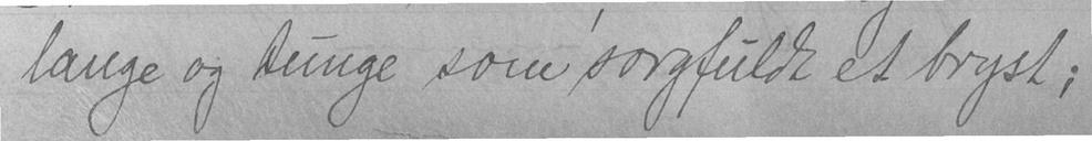lange og tunge som sorgfuldt et bryst;
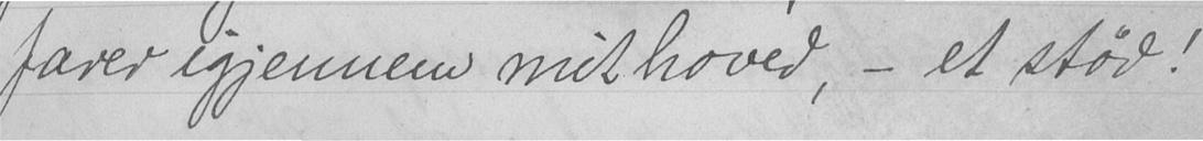farer igjennem mit hoved, - et stød!
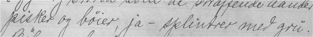pisker og bøier, ja - splintrer med gru.
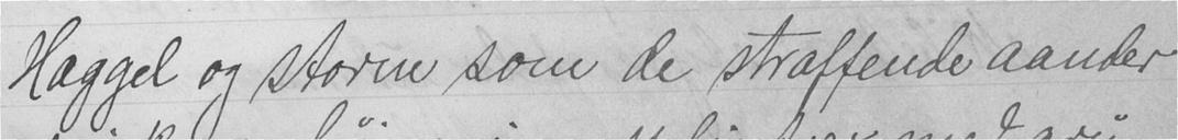Haggel og storm som de straffende aander
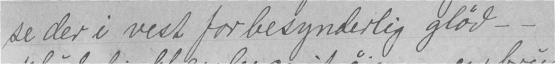se der i vest for besynderlig glød - -
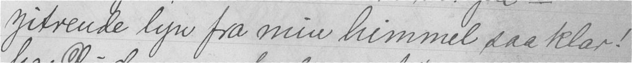zitrende lyn fra min himmel saa klar!
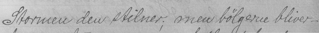Stormen den stilner; men bølgerne bliver -
